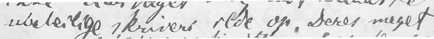ubeleilige skriveri ilde op. Deres meget
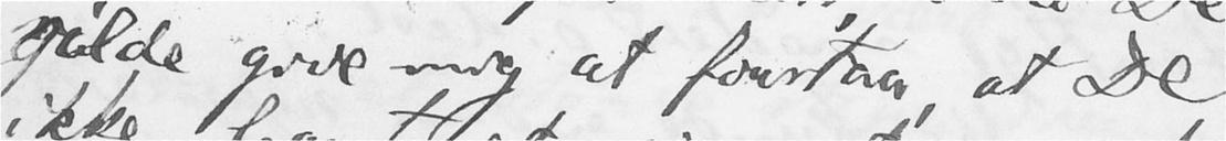vilde give mig at forstaa, at De
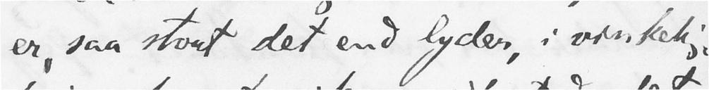er, saa stort det end lyder, i virkelig-
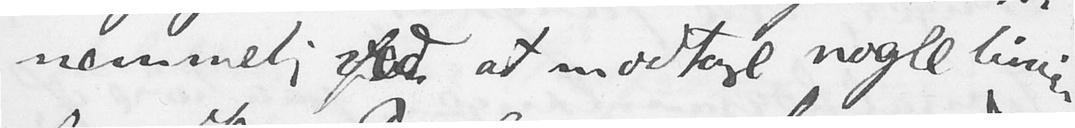nemmelig ved, at modtage nogle linier
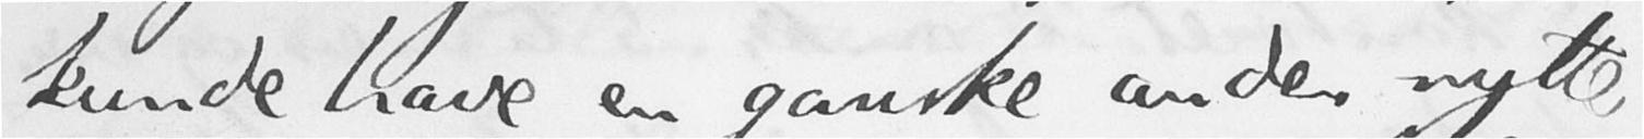kunde have en ganske anden nytte
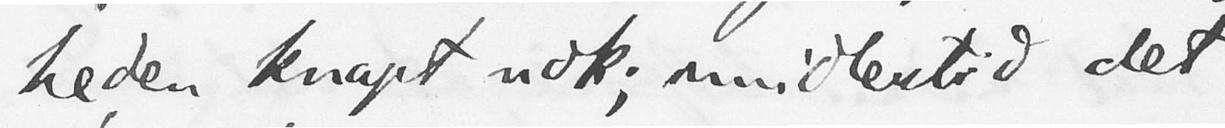heden knapt nok; imidlertid det
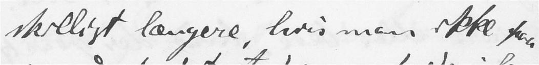skilligt længere, hvis man ikke paa
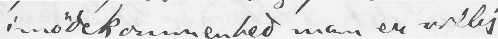imødekommenhed, man er villig
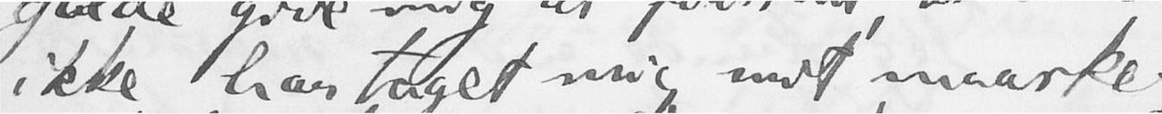ikke har taget mig mit maaske
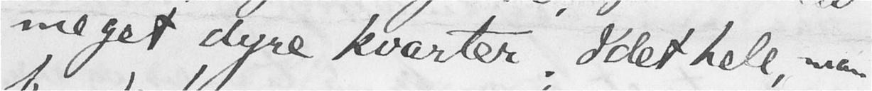meget dyre kvarter. Idet hele, man
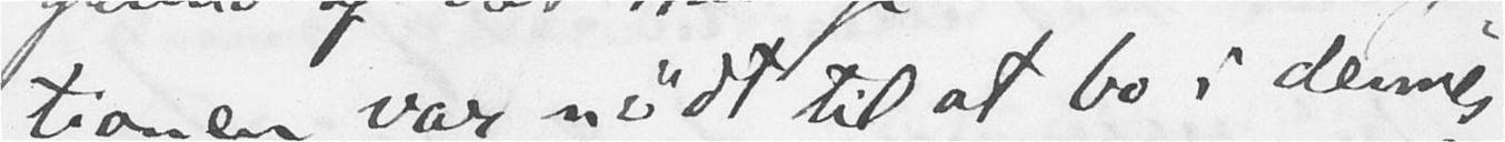tionen var nødt til at bo i dennes
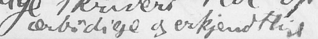ærbødige og erkjendtlige
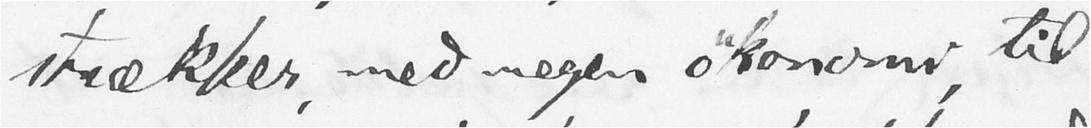strækker, med megen økonomi, til.
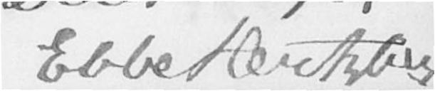Ebbe Hertzberg
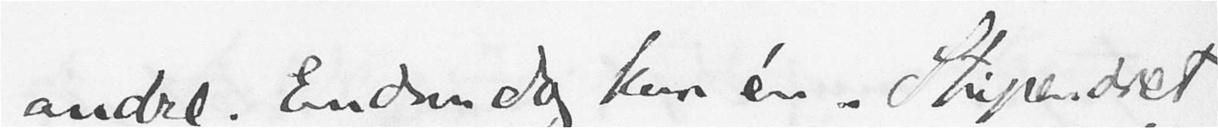andre. Endnu dog kun én. Stipendiet
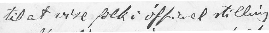til at vise folk i officiel stilling.
